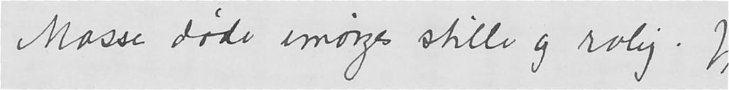Mosse døde imorges stille og rolig. Jeg
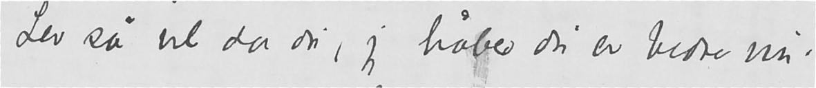Lev si vel da du, jeg haber du er bedre nu.
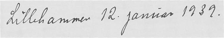Lillehammer 12. januar 1939.
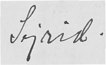Sigrid
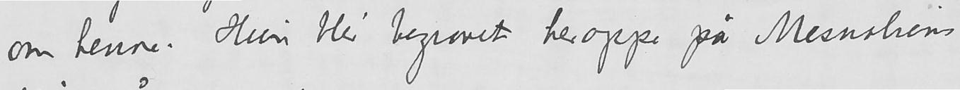om henne. Hun ble begravet heroppe pa Mesnali
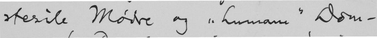sterile Mødre og "humane" Dom-
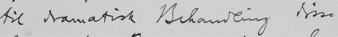til dramatisk Behandling disse
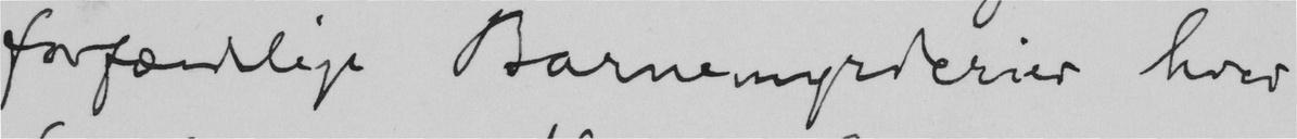forfærdelige Barnemyrderier hver
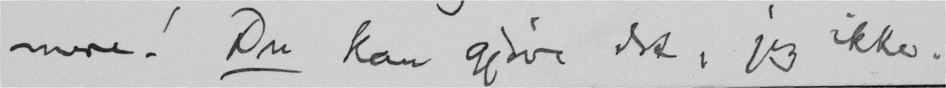mere! Du kan gjøre det, jeg ikke.
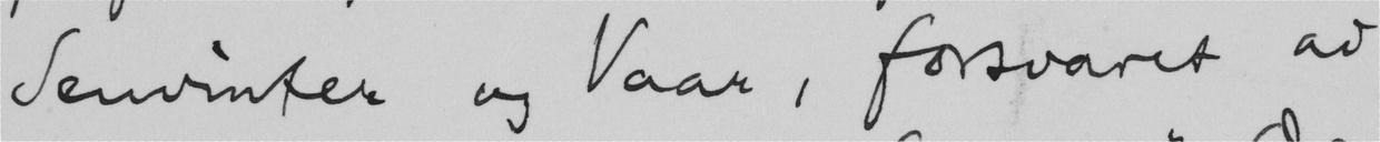Senvinter og Vaar, forsvaret av
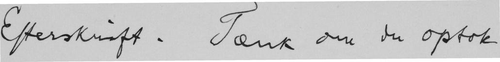Efterskrift. Tænk om du optok
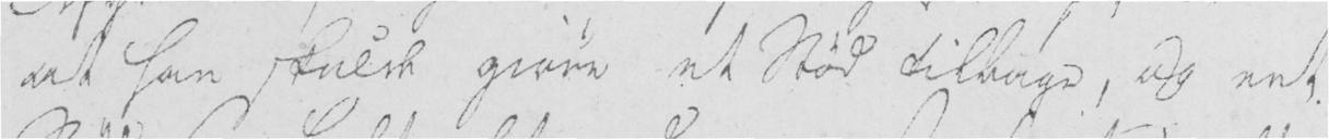at han skulde giøre et Stød tilbage, og eet
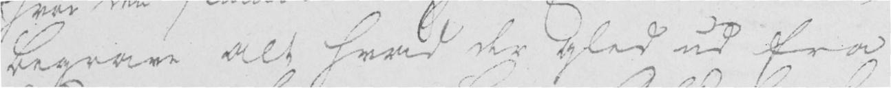begrave alt hvad der gled ud fra
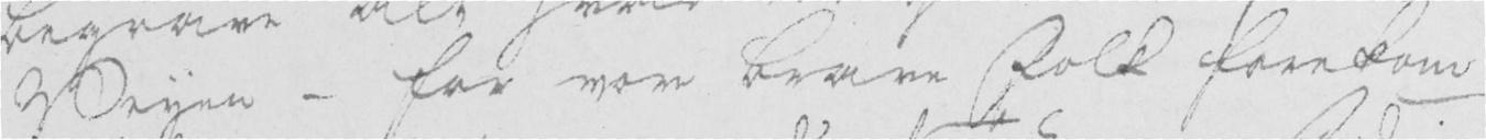Veyen - for vore brave Folk forekom
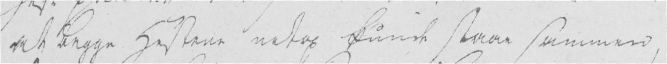at begge Hestene netop kunde staae sammen,
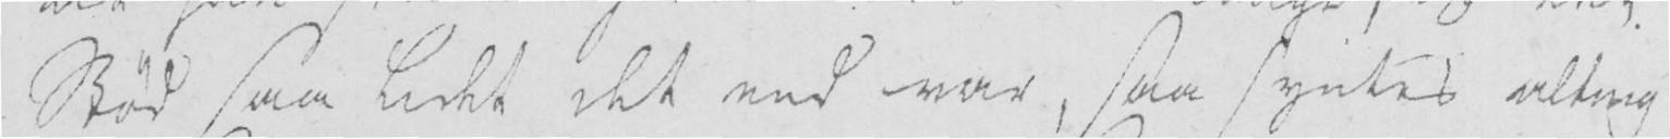Stød saa lidet det end var, saa syntes alting
